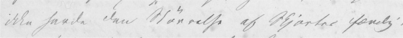ikke havde den Størrelse af Skjorter færdig.
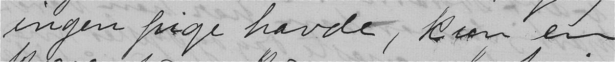ingen pige havde, kun en
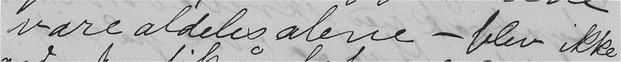være aldeles alene - blev ikke
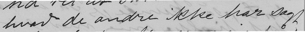hvad de andre ikke har sagt
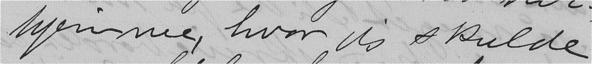hjemme, hvor jeg skulde
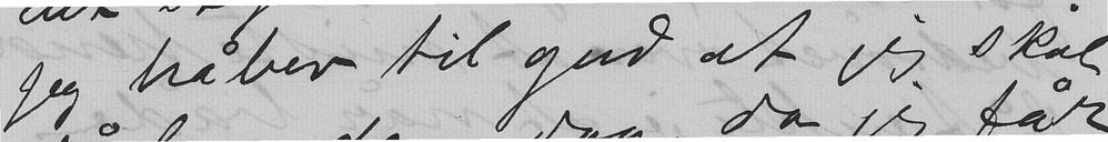Jeg håber til gud at jeg skal
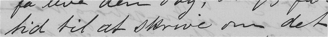tid til at skrive om det
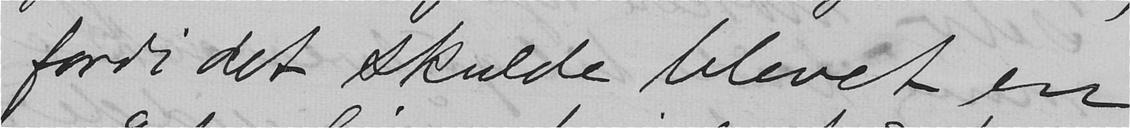fordi det skulde blevet en
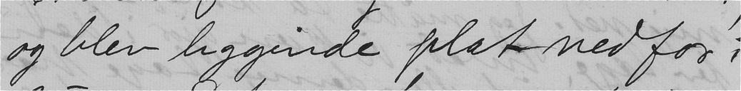og blev liggende plat nedfor i
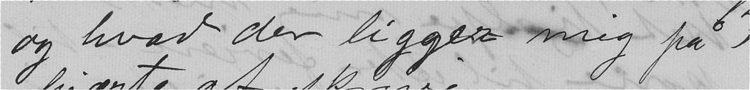og hvad der ligger mig på
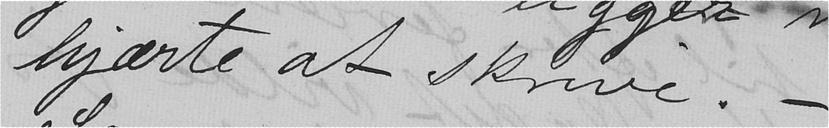hjærte at skrive. -
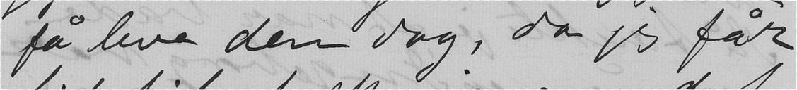få leve den dag, da jeg får
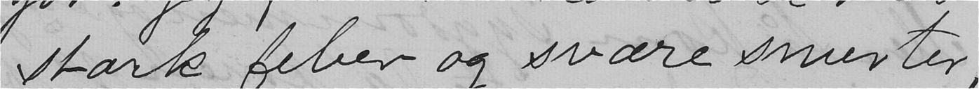stærk feber og svære smerter,
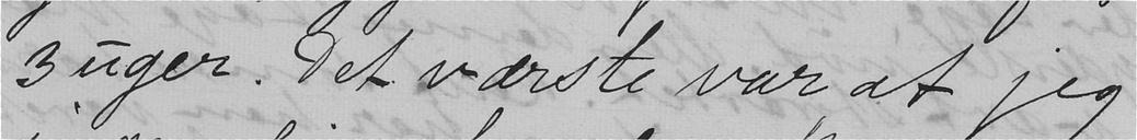3 uger. Det værste var at jeg
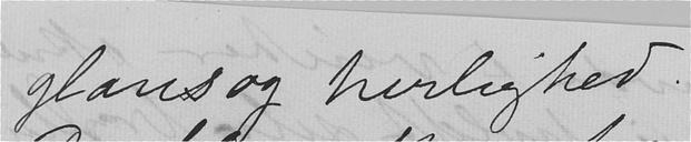glans og herlighed.
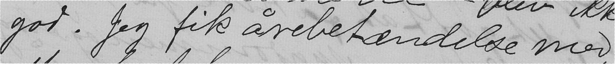god. Jeg fik årebetændelse med
